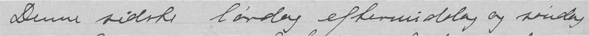Denne sidste lørdag eftermidelag og søndag
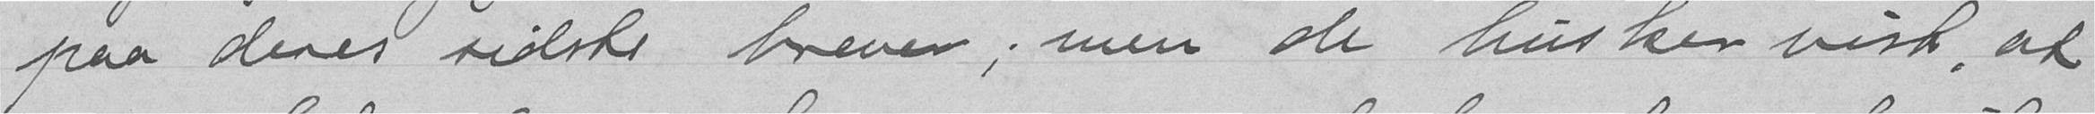paa deres sidste brever; men de husker vist at
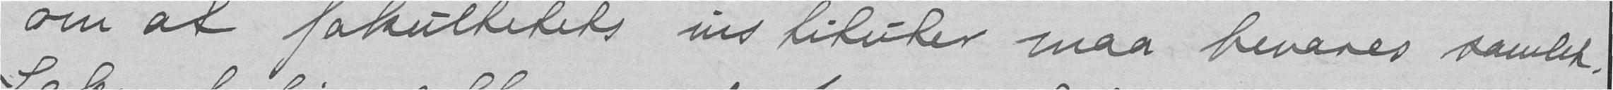om at fakultetets instituter maa bevares samlet.
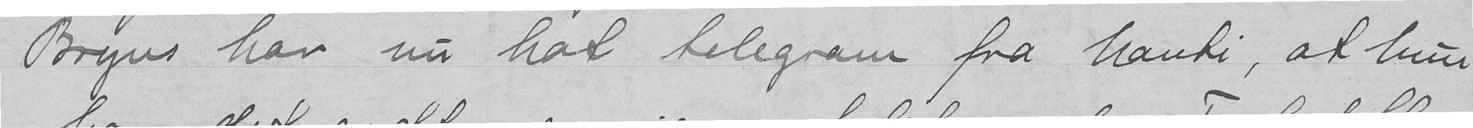Bryns har nu hat telegram fra Nanti, at hun
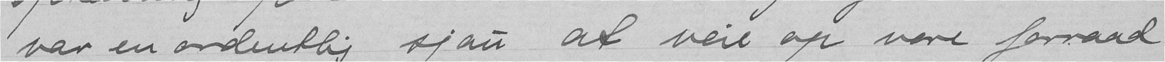var en ordentlig sjau at veie op vore forraad
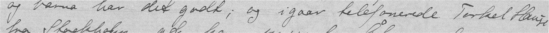og barna har det godt; og igaar telefonerede Torkel Hause
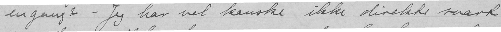engang? - Jeg har vil kanske ikke direkte svart
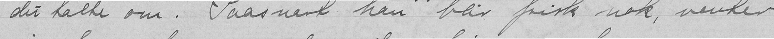du talte om. Saasnart han blir frisk nok, venter
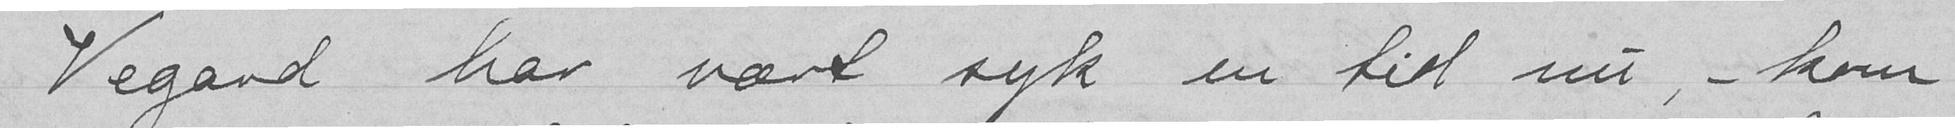Vegard har vært syk en tid nu ,- kom
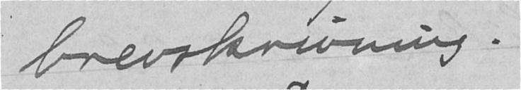brevskrivning.
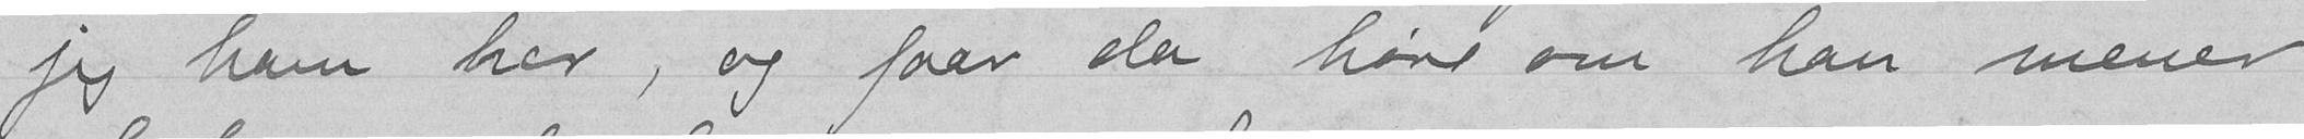jeg ham her, og faar da høre om han mener
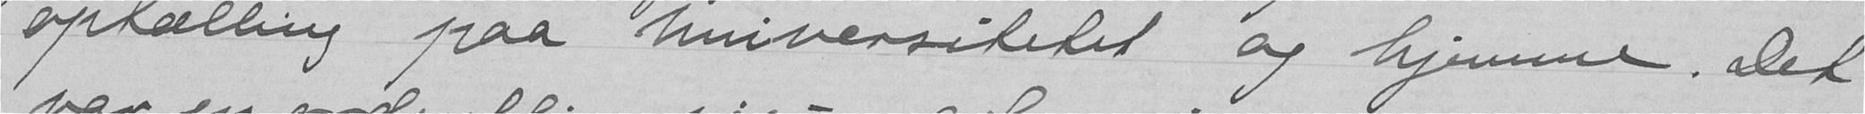optælling paa Universitetet og hjemme. Det
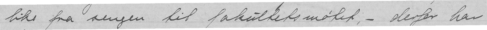like fra sengen til fakultetsmøtet, - derfor har
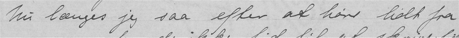Nu længes jeg saa efter at høre lidt fra
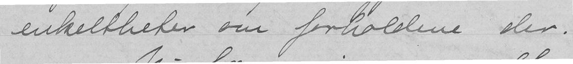enkeltheter om forholdene der.
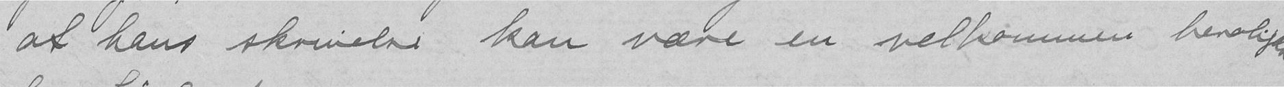at hans skrivelse kan være en velkommen beroligelse
